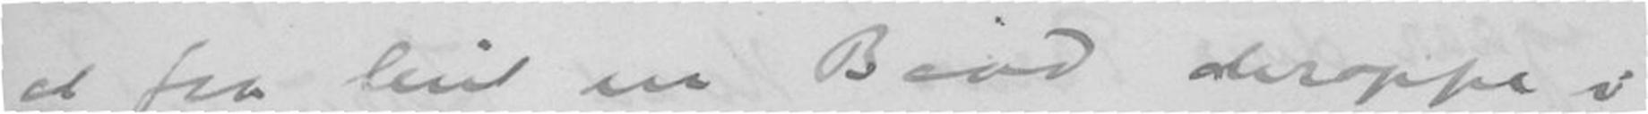at faa lånt en Baad deroppe i
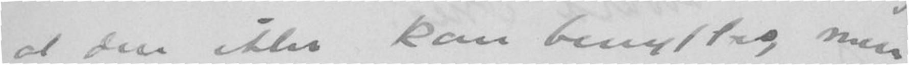at den ikke kan benyttes, men
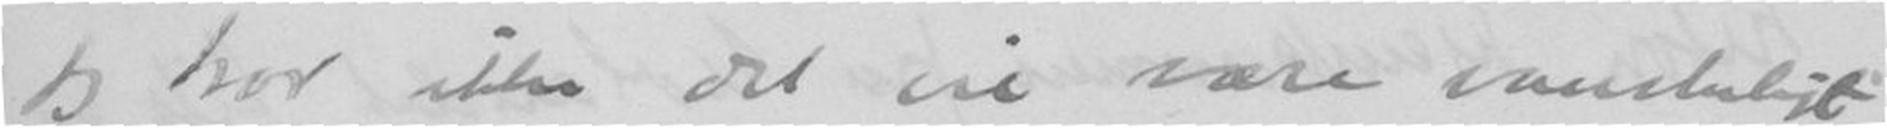jeg tror ikke det vil være vanskeligt
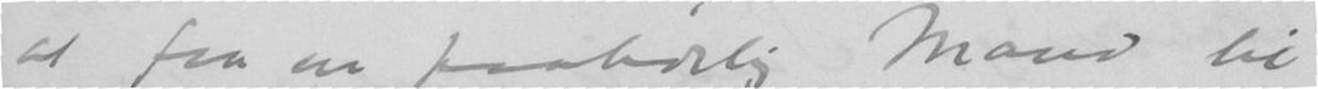at faa en paalidelig Mand til
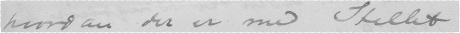hvordan der er med Stellet
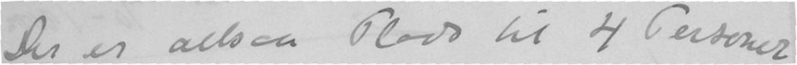Der er altsaa Plads til 4 Personer
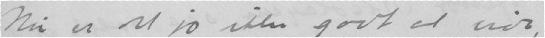Nu er det jo ikke godt at vide,
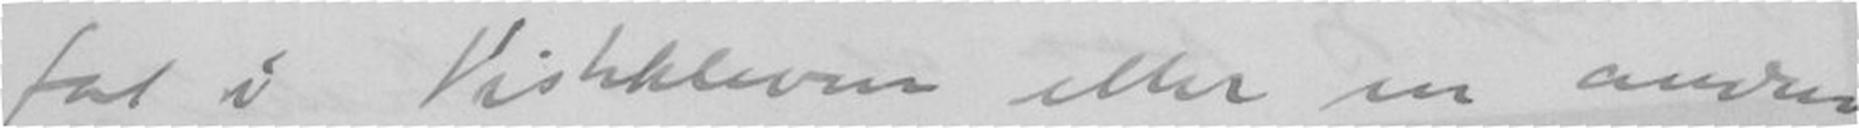fat i Viskkleven eller en anden
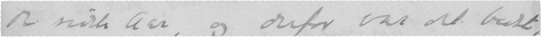de sidste Aar, og derfor var det bedst,
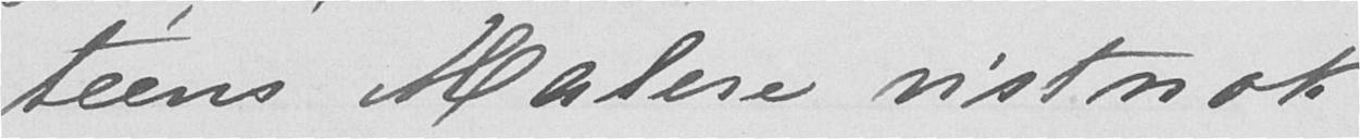téens Malere vistnok
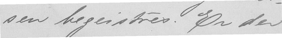sen begeistres. Er der
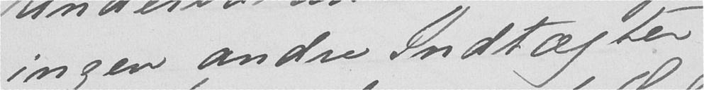ingen andre Indtægter
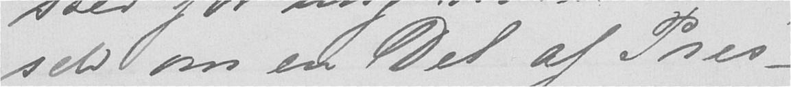selv om en Del af Pres-
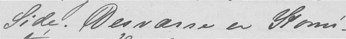Side. Desværre er Komi-
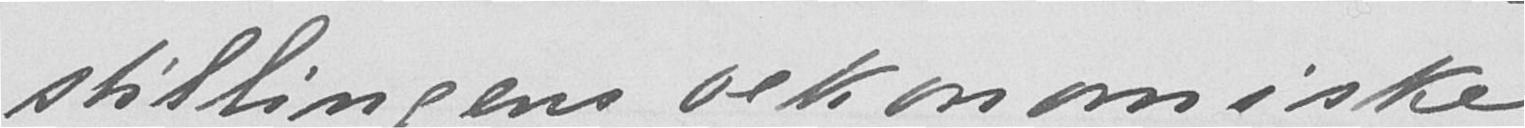stillingens oekonomiske
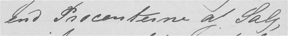end Procenterne af Salg,
